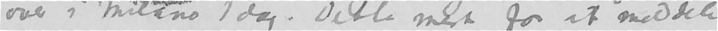over i Milano i dag. Dette mest for at meddele
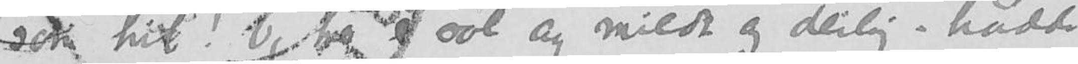som hit! Og her er sol og mildt og deilig – hadde
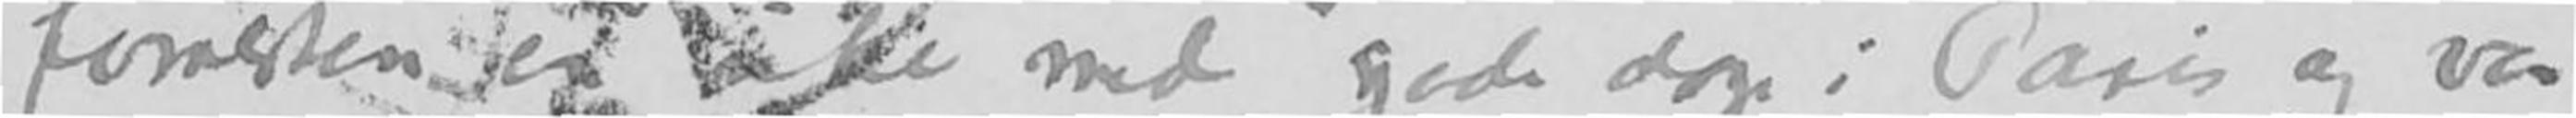forresten en uke med gode dager i Paris og var
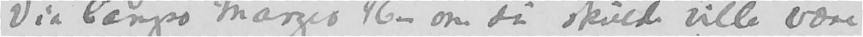Via Campo Marzio 46 – om du skulde ville være
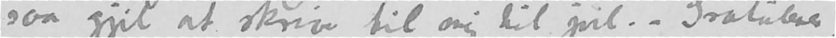saa gjil at skrive til mig til jul. – Gratulerer
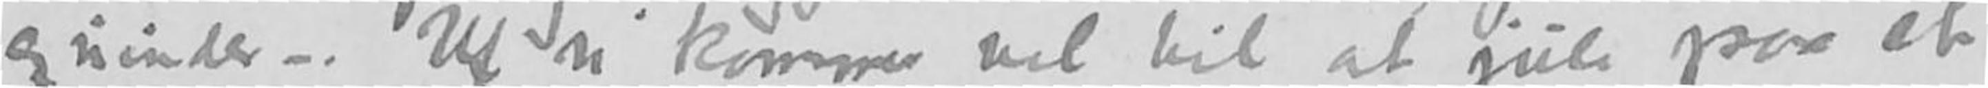qvinder –. Uf vi kommer vel til at jule paa et
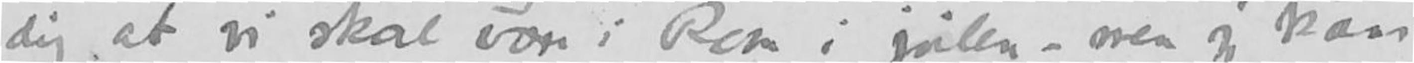dig at vi skal være i Rom i julen – men jeg kan
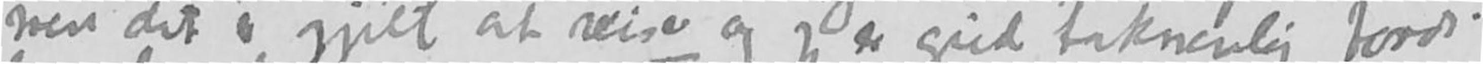men det er gjilt at reise og jeg er gud taknemlig fordi
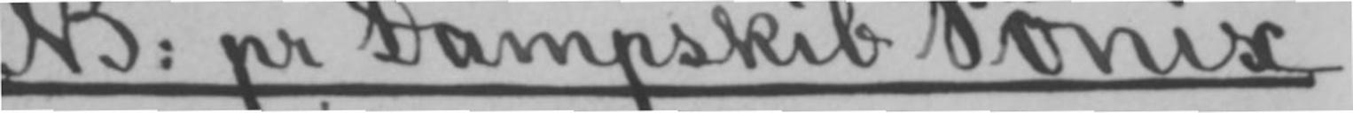NB: pr Dampskib Fønix.
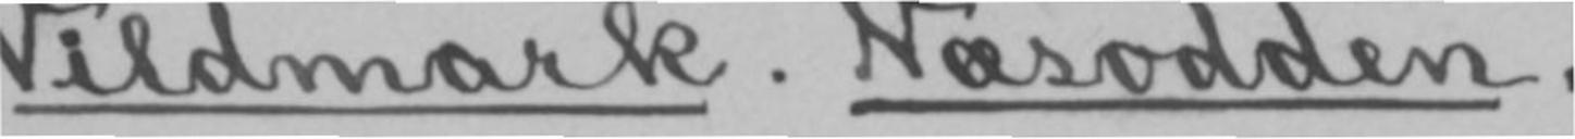Vildmark. Næsodden.
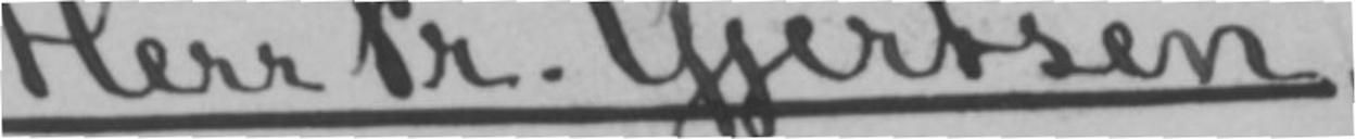Herr Fr. Gjertsen,
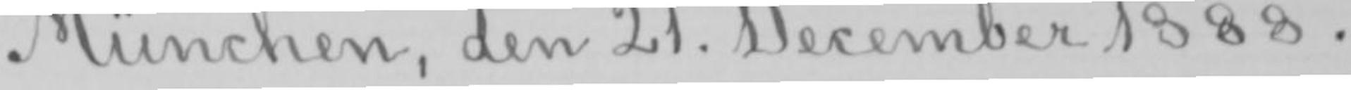München, den 21. December 1888.
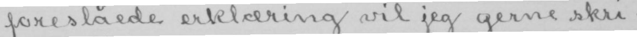foreslåede erklæring vil jeg gerne skri-
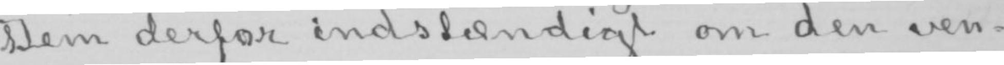Dem derfor indstændigt om den ven-
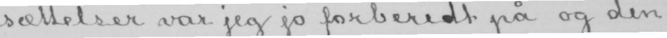sættelser var jeg jo forberedt på og den
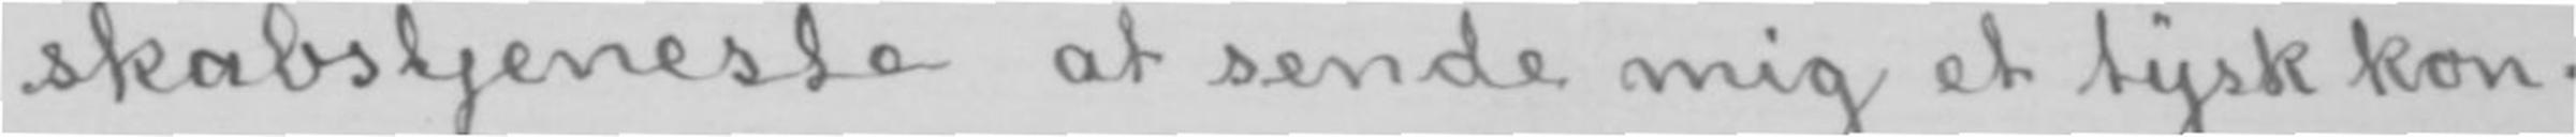skabstjeneste at sende mig et tysk kon-
